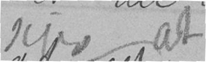siges at
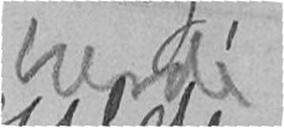burde
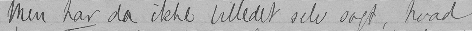Men har da ikke billedet selv sagt, hvad
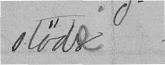støde
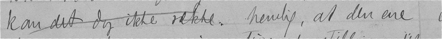kan det dog ikke række. Nemlig, at den ene
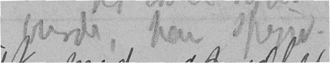burde, han sprger.
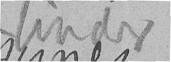finder
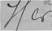Her
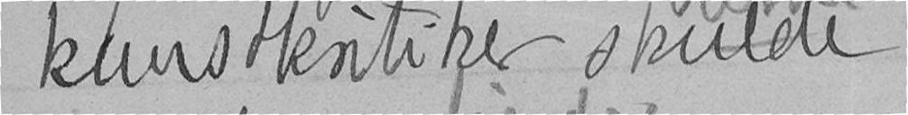kunstkritiker skulde
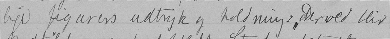lige figurers udtryk og holdning: "Derved blir
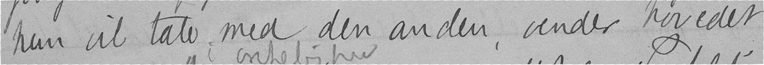hun vil tale med den anden, vender hovedet
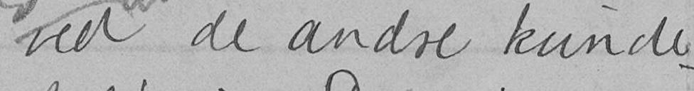ved de andre kvinde-
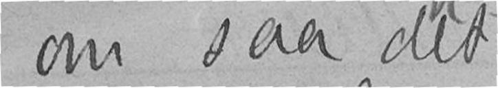om saa det
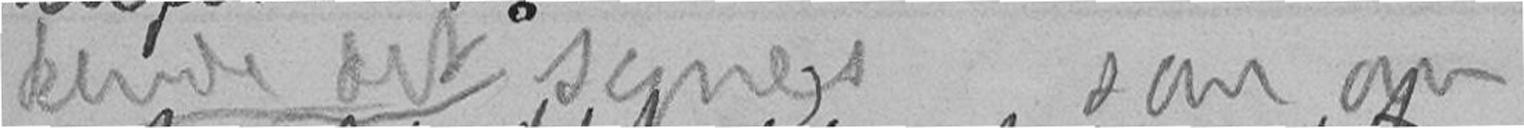kunde det synes som om
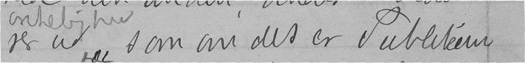ser ud, som om det er Publikum
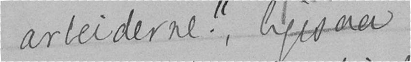arbeiderne.", ligesaa
